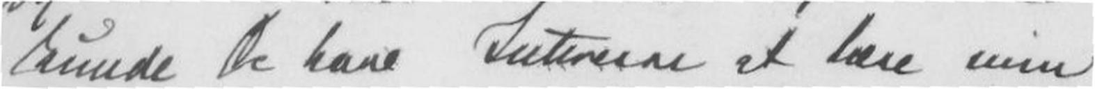kunde De have Interesse at lære min
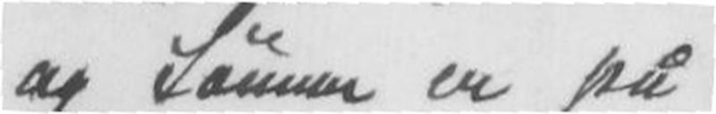og Sønnen er på
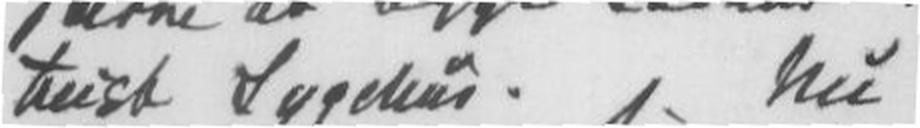trist Sygehus. Nu
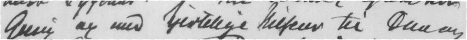Grieg og med hjertelige Hilsener til Dem og
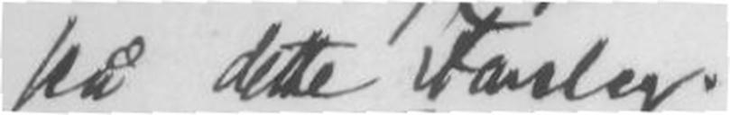på dette forslag.
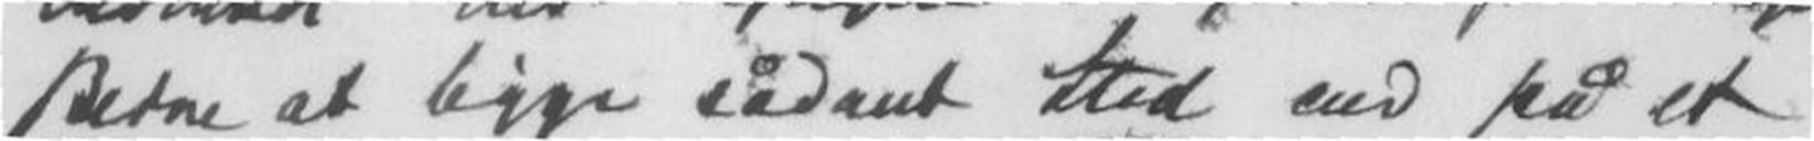Betal at ligge sådant Sted end på et
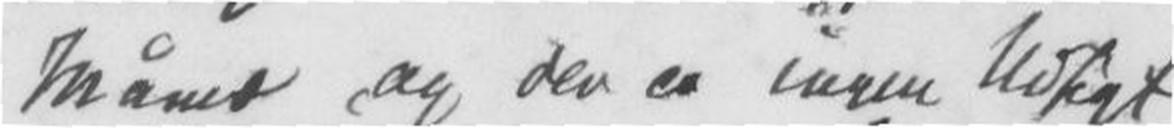Måned, og der er ingen Udsigt
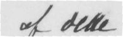af dette
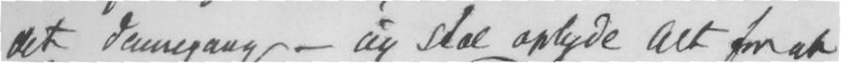det dennegang - og skal aplyde alt for at
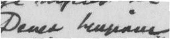Deres hengivne
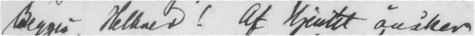Begges Helbred! Af Hjertet ønsker
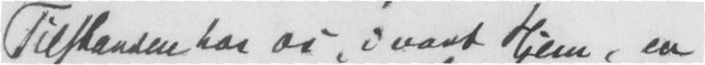Tilstanden har vi, i vart Hjem, en
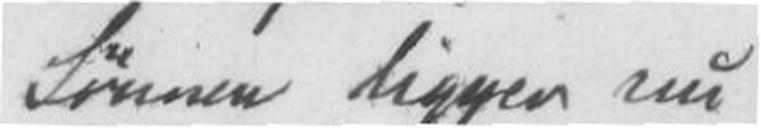Sønnen ligger nu
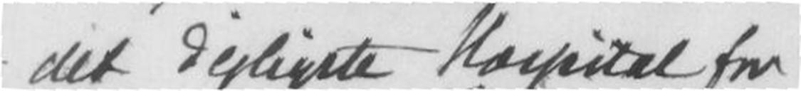ind det dejligste Hospital for
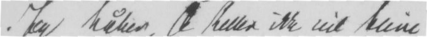. Jeg håber, De heller ikke vil {??]
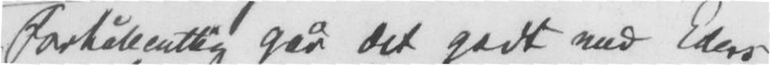Forhåbentlig går det godt med Eder
